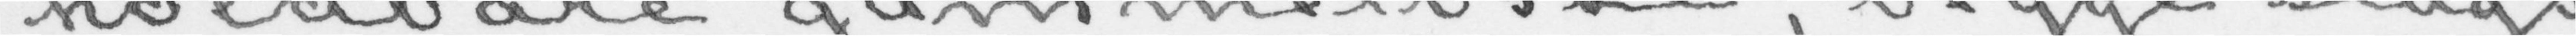holdbare gammeloste, begge slags
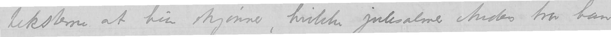hun skjønner, hvilken julesalme Anders tror han
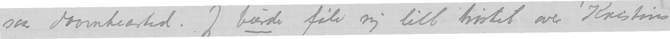saa dovenhearted. Jeg burde føle mig litt trøstet over «Kristins»
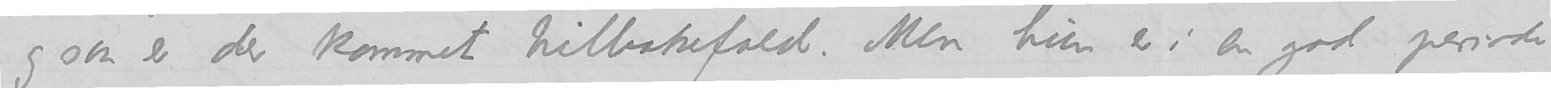og saa er der kommet tilbakefald.  Men hun er i en god periode –
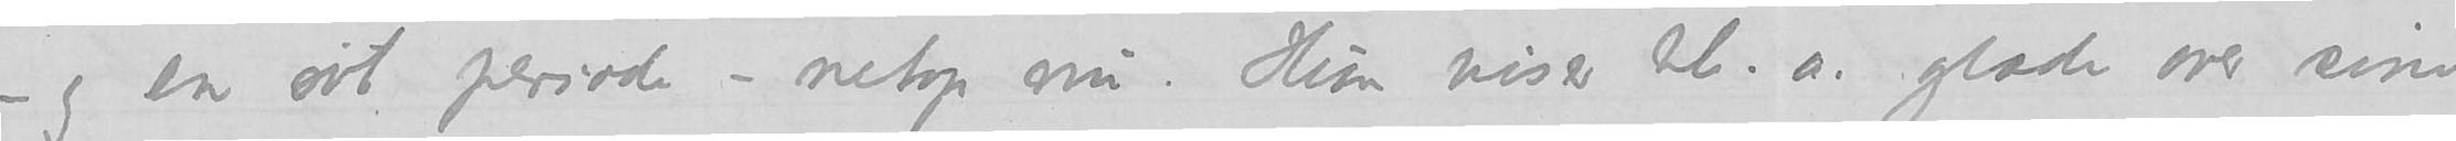og en søt periode – netop nu.  Hun viser bl.a. glæde over sine
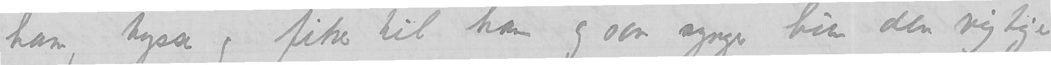ham, kysser og fiker til ham og saa synger hun den rigtige
